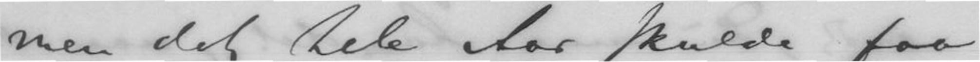men det hele Aar skulde faa
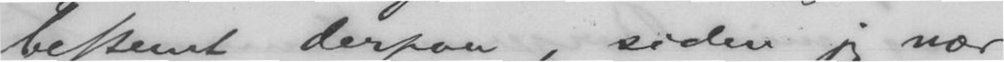bestemt derpaa, siden jeg nær
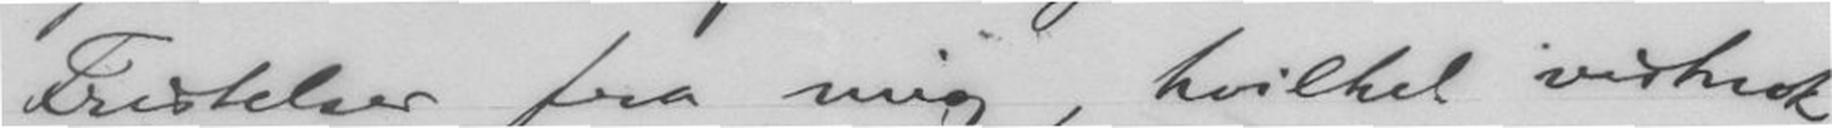Fristelser fra mig, hvilket vistnok
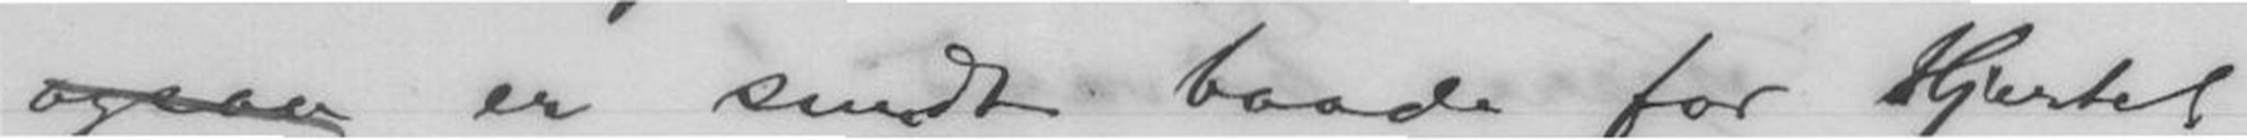ogsaa er sundt baade for Hjertet
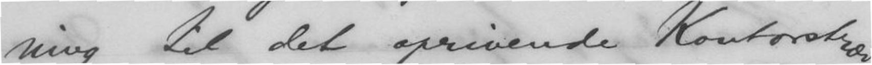ning til det oprivende Kontorstræv.
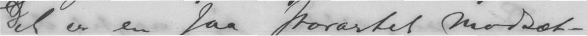Det er en saa storartet Modsæt-
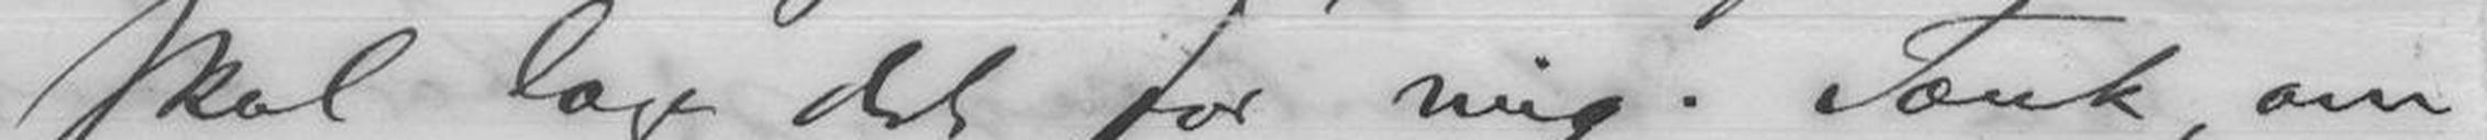skal lage det for mig. Tænk, om
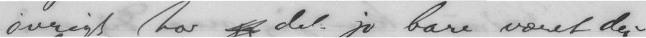øvrigt har jeg det jo bare været dei-
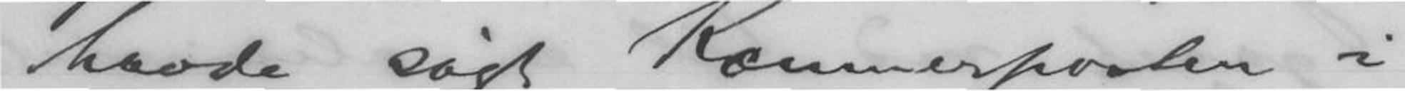havde søgt Kæmnerposten i
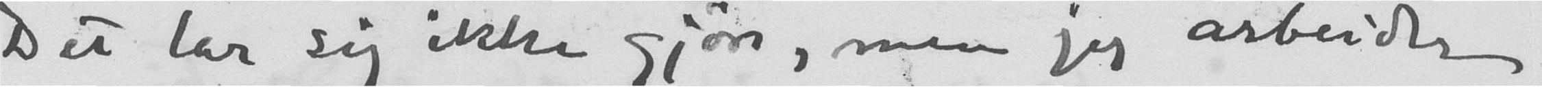Det lar sig ikke gjøre, men jeg arbeider
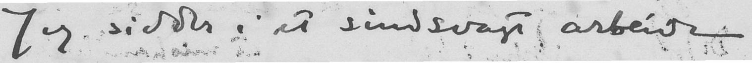Jeg sidder i et sindsvagt arbeide
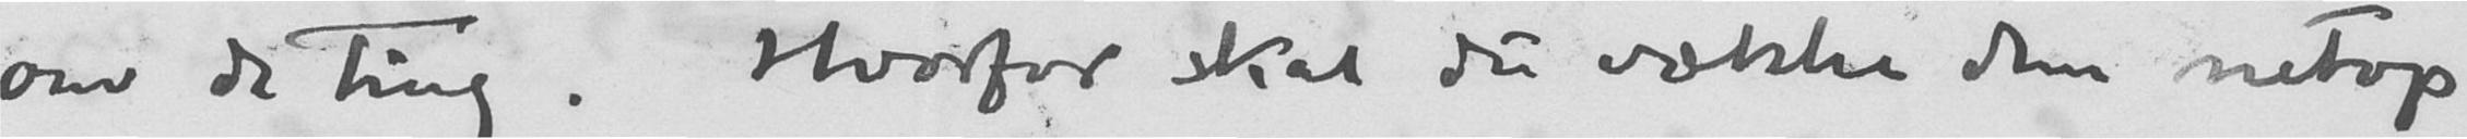om de ting. Hvorfor skal du vække dem netop
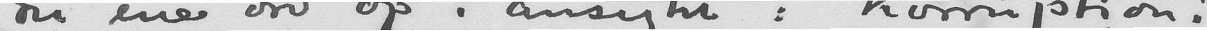det ene ord op i ansigtet: korruption!
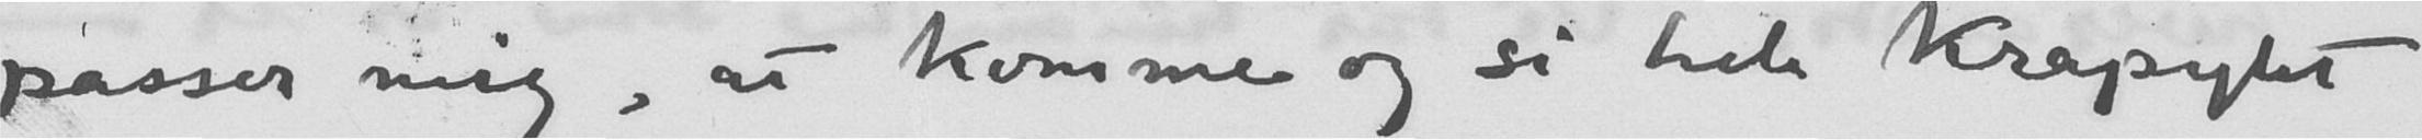passer mig, at komme og si hele krapylet
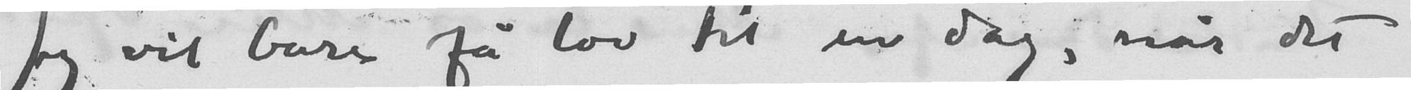Jeg vil bare få lov til en dag, når det
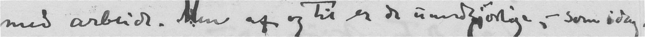med arbeide. Men af og til er de umedgjørlige, - som idag.
